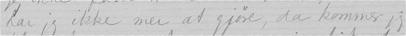har jeg ikke mer at gjøre, da kommer jeg
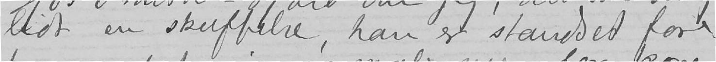lidt en skuffelse, han er standset fore-
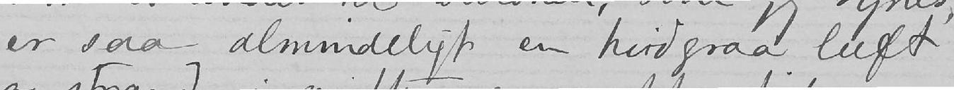er saa almindeligt en hvidgraa luft
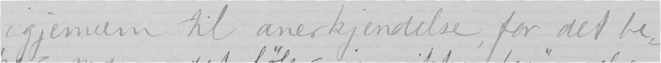igjennem til anerkjendelse, for det be-
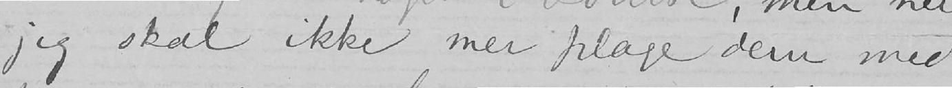jeg skal ikke mer plage dem med
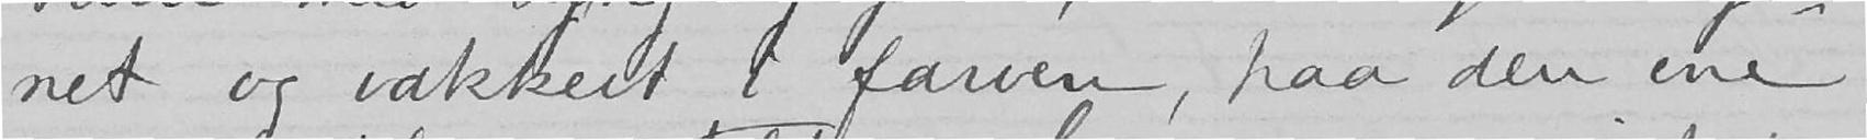net og vakkert i farven, paa den ene
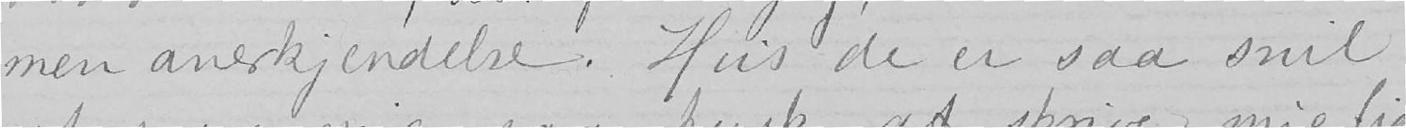men anerkjendelse. Hvis de er saa snil
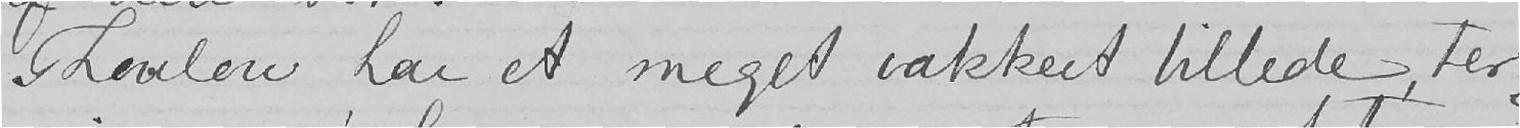Thoulow har et meget vakkert billede, ter-
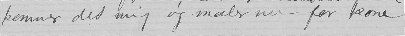kommer det mig og maler nu for kone
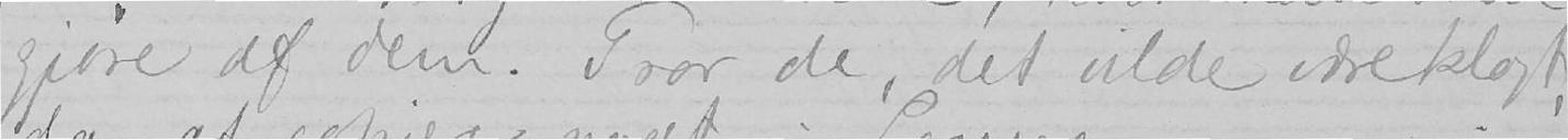gjøre af dem. Tror de, det vilde være klogt,
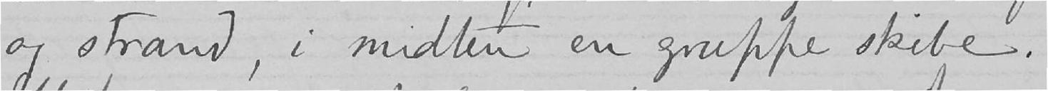og strand, i midten en gruppe skibe.
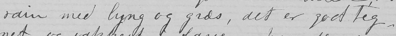rain med lyng og græs, det er godt teg-
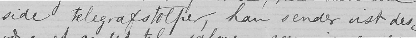side telegrafstolper, han sender vist des-
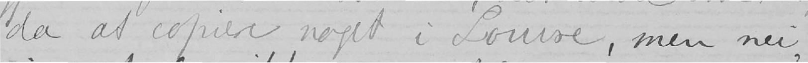da at copiere noget i Louvre, men nei
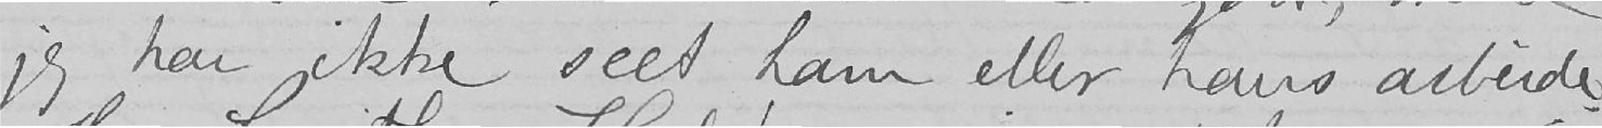jeg har ikke seet ham eller hans arbeide.
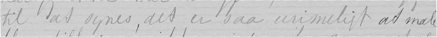til at synes, det er saa urimeligt at male
